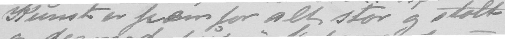Kunst er fremfor alt stor og stolt
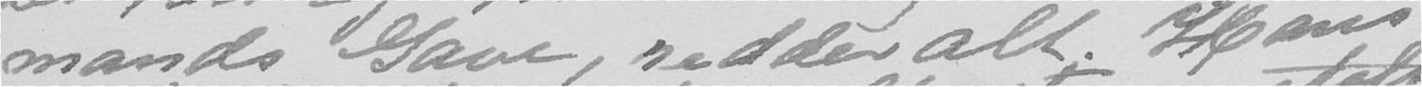mands Gave, redder alt. Hans
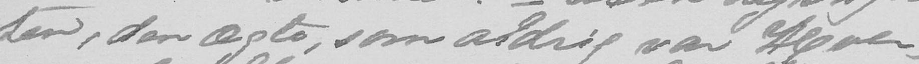ten, den ægte, som aldrig var Hver-
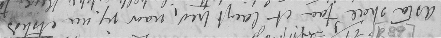Asta skal faa et langt brev, naar jeg nu etsteds
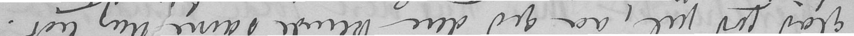glad god jul, aa gid dere kunde savne mig lidt.
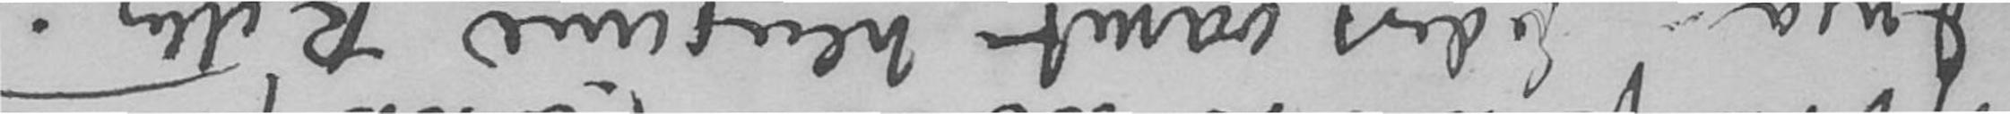Inga. Eders varmt hengivne Kitty.
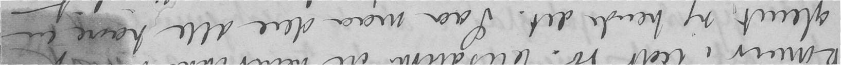glemt, sig hende det. Saa maa dere alle have en
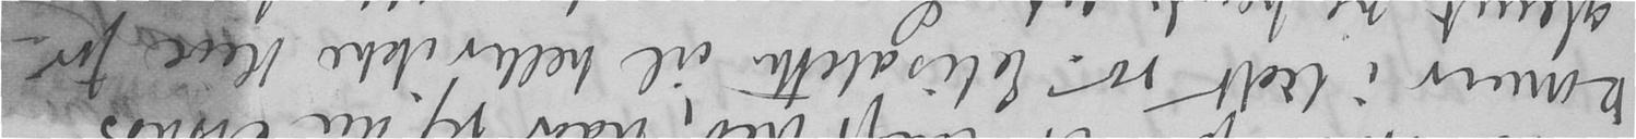kommer i lidt ro. Elisabeth vil heller ikke blive for-
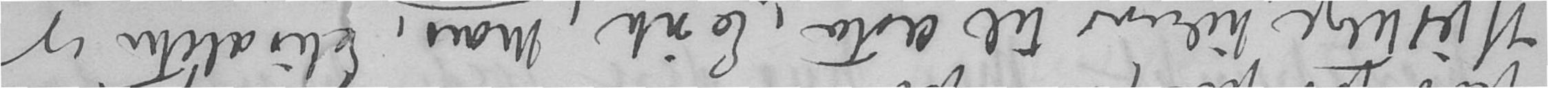Hjertelige hilsen til Asta, Erik, Mons, Elisabeth og
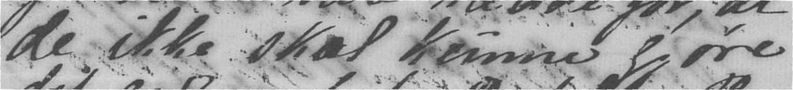de ikke skal kunne gjøre
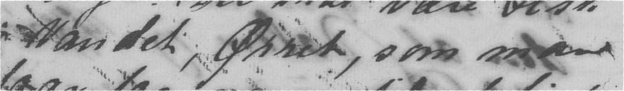i Vandet, Ørret, som man
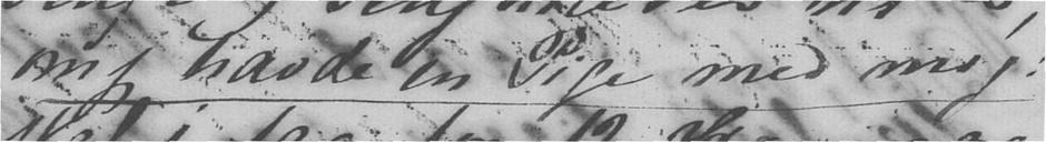om jeg havde en Pige med mig!
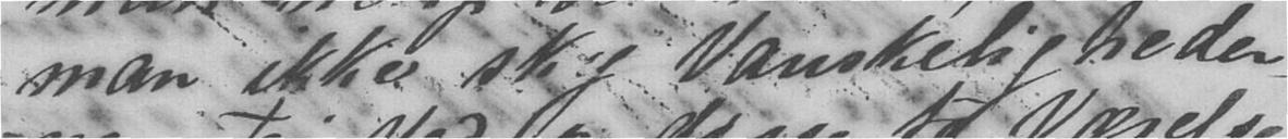man ikke sky Vanskeligheder-
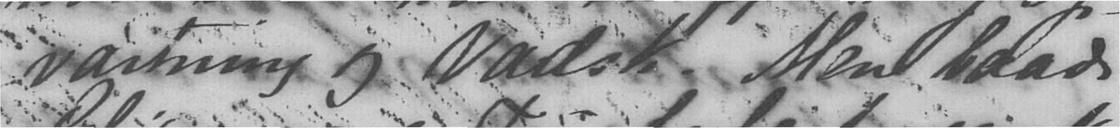vartning og Vadsk. Men baade
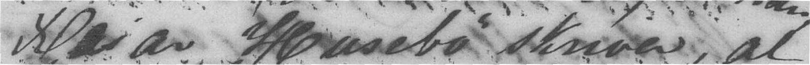Reiar Husebø skriver, at
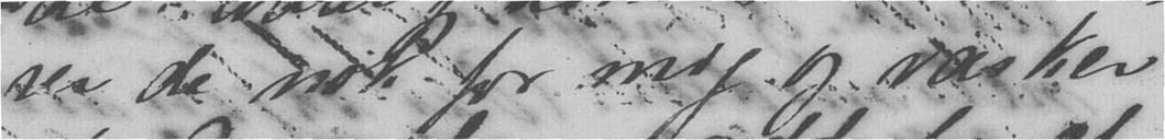rer de nok for mig og vasker
